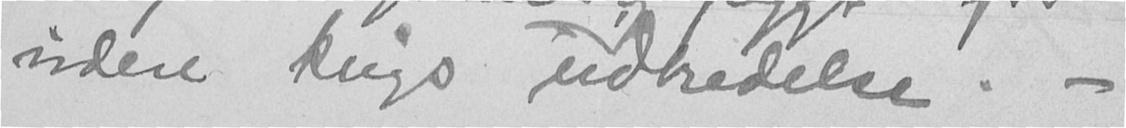videre krigs udbredelse. -
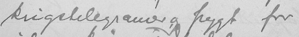krigstelegramer og frygt for
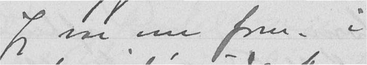Jeg var om form. i
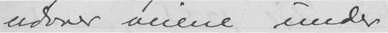udover veiene under
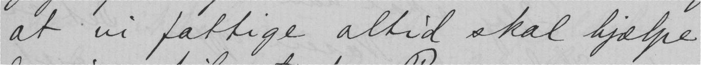at vi fattige altid skal hjælpe
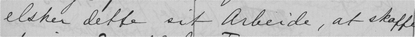elsker dette sit arbeide, at skaffe
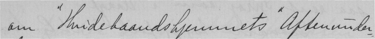om "Hvidebaandshjemmets" aftenunder-
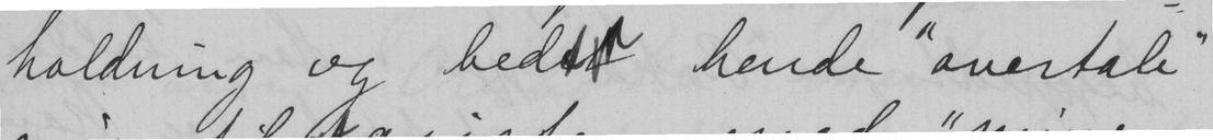holdning og bedtt hende "overtale"
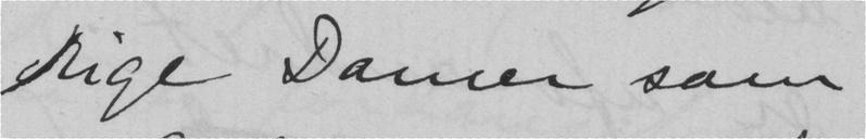rige Damer som
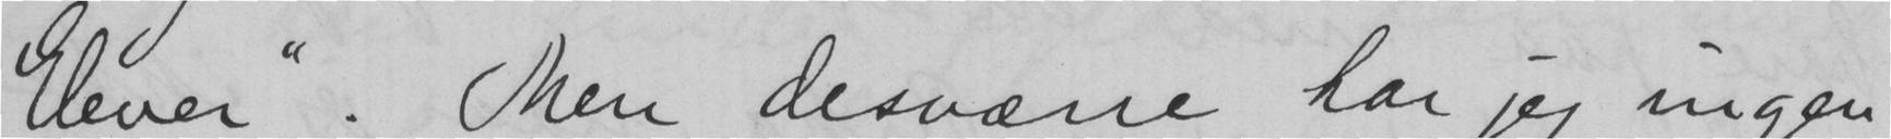Elever". Men desværre har jeg ingen
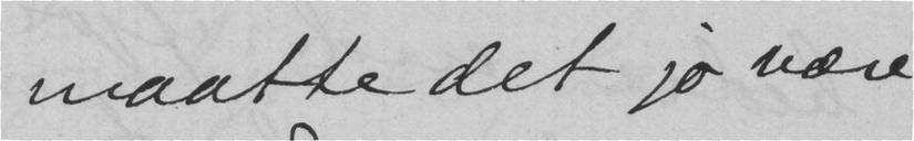maatte det jo være
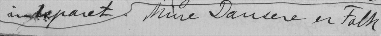indsparet. Mine Dansere er Folk
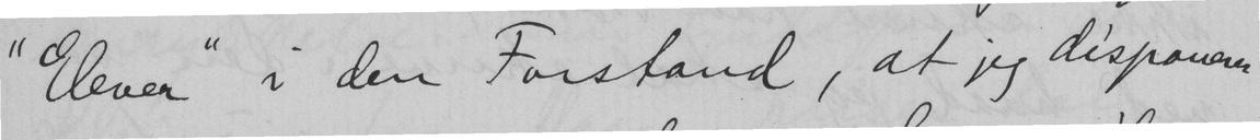"Elever" i den Forstand, at jeg disponerer
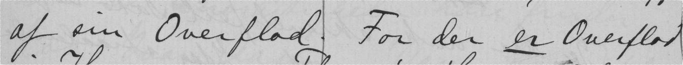af sin Overflod. For der er Overflod
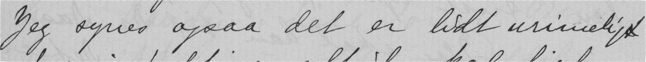Jeg synes ogsaa det er lidt urimeligt
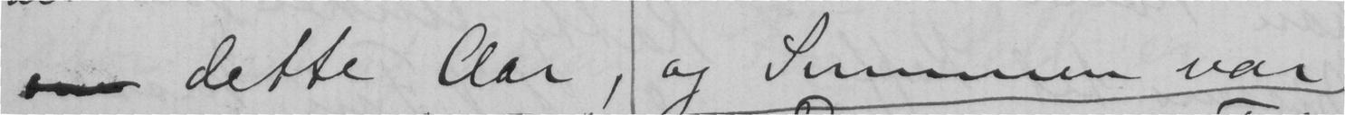dette Aar; og Summen var
